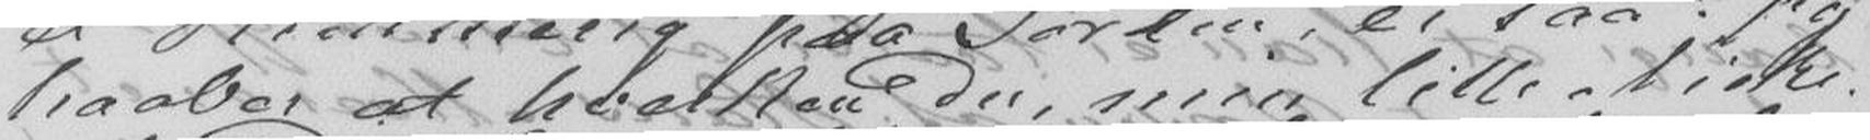haaber at hverken Du, min lille Niske
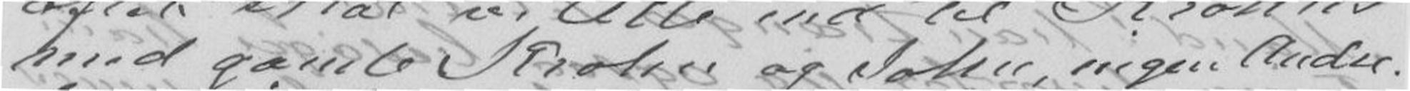med gamle Krohn og John, ingen Ander.
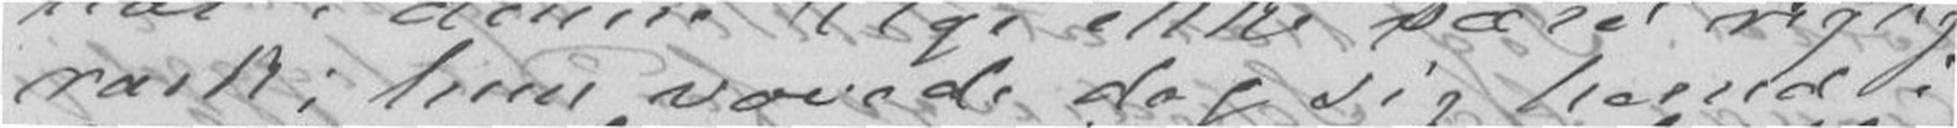rask; hun vovede dog sig herud i
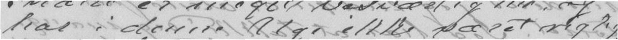har i denne Uge ikke været rigtig
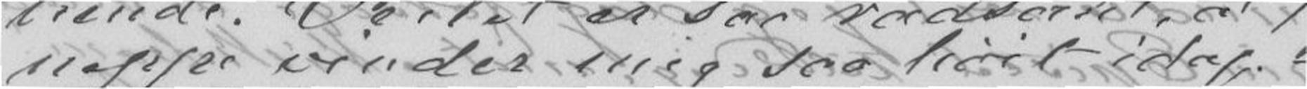neppe vinder mig saa høit idag.
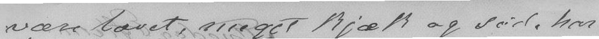være lovet, meget kjæk og sød, har
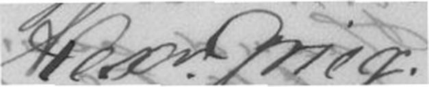Alexr Grieg
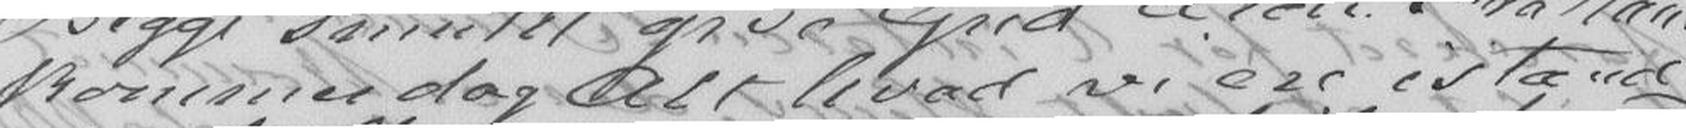kommer dog Alt hvad vi ere istand
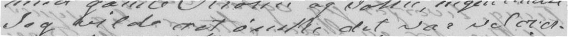Jeg vilde ret ønske det var velover-
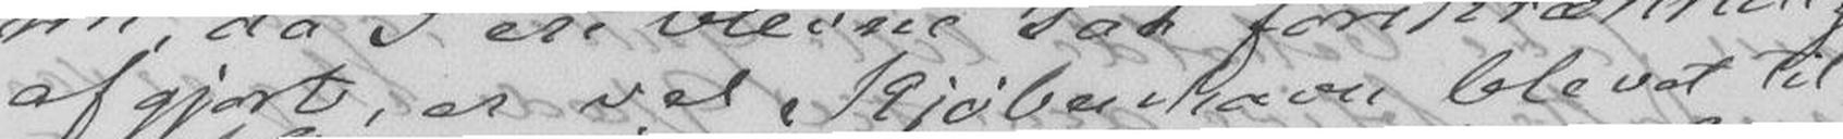afgjort, er vel Kjøbenhavn blevet til
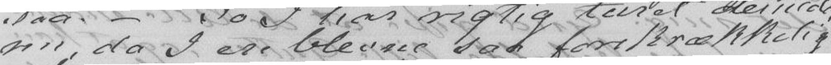nu, da I ere blevne saa forskrækkelig
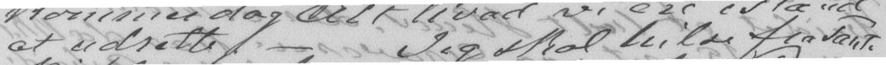at udrette. - Jeg skal hilse fra Tante
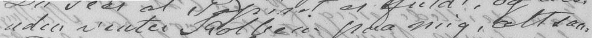uden venter Kolbein paa mig, altsaa
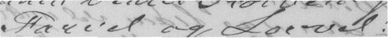Farvel og Levvel!
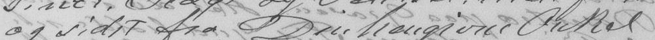og sidst fra Din hengivne Onkel
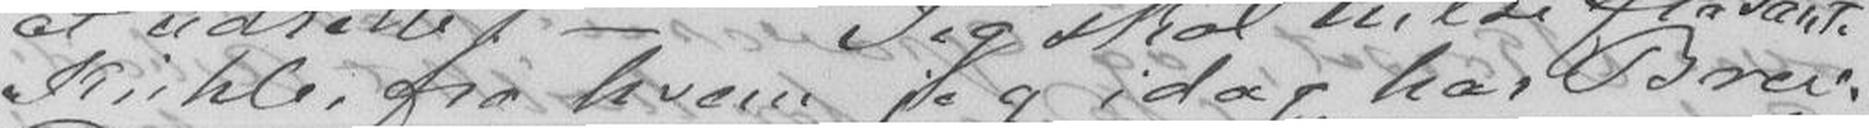Kühle, fra hvem jeg idag har Brev.
